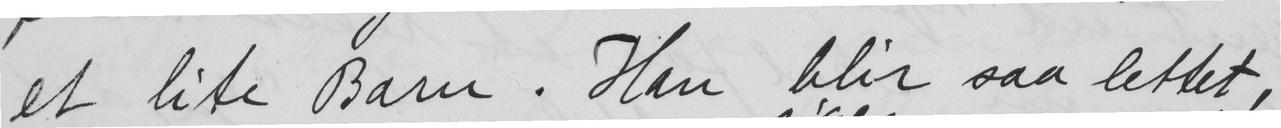et lite Barn. Han blir saa lettet,
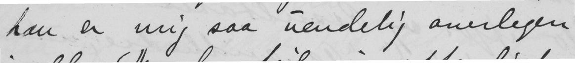han er mig saa uendelig overlegen
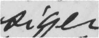siger
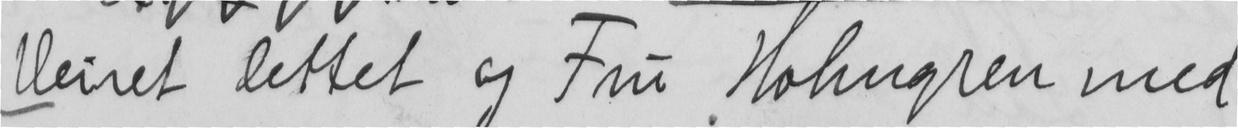Veiret lettet og Fru Holmgren med
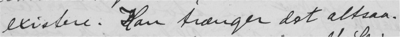existere. Han trænger det altsaa.
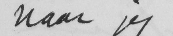naar jeg
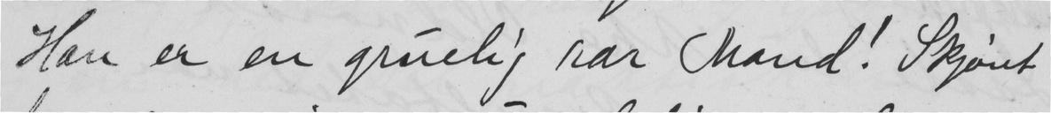Han er en gruelig rar Mand! Skjønt
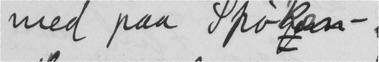med paa Spøken -
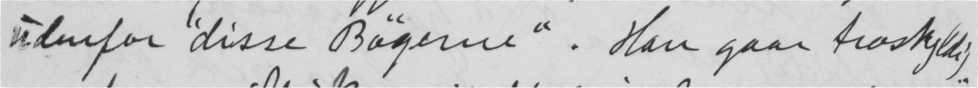udenfor "disse Bøgerne". Han gaar troskyldige
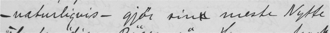- naturligvis - gjør sin meste Nytte
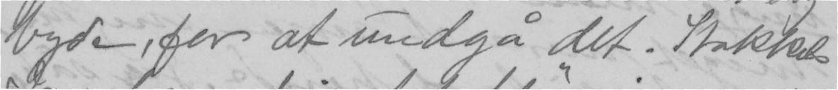byde, for at undgå det. Stakkels
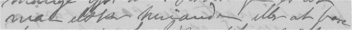man elsker hianden eller at bare
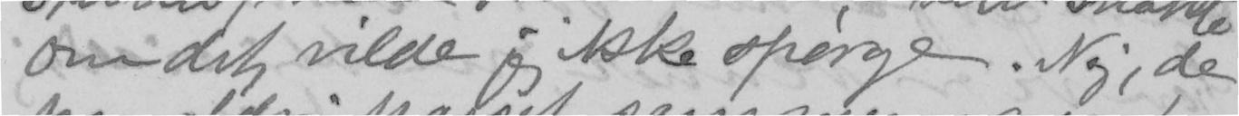om det vilde jeg ikke spørge. Nej, de
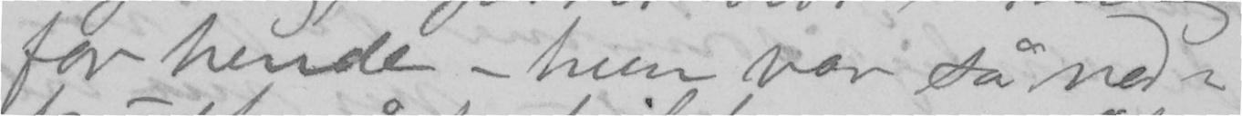for hende – hun var så ned-
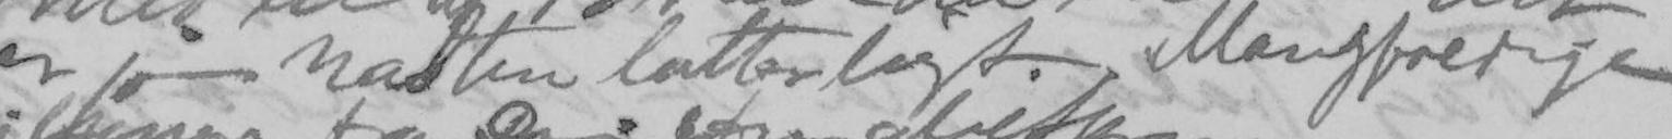er jo næsten latterligt. Mangfoldige
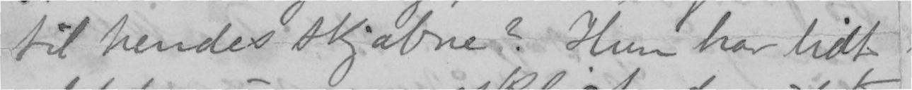til hendes skjæbne? Hun har lidt
